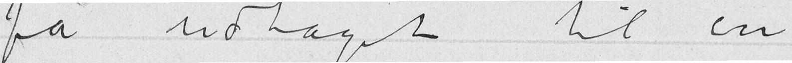få udtaget til en
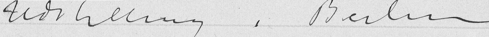Udstilling i Berlin
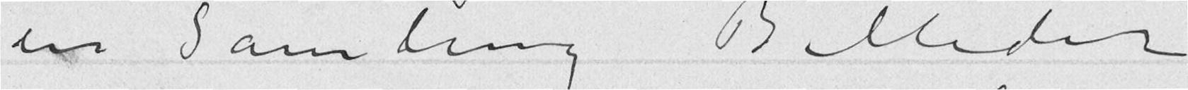en Samling Billeder
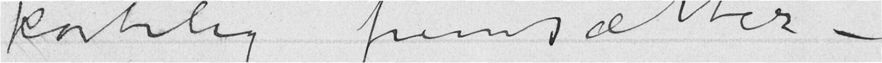kortelig fremsætter –
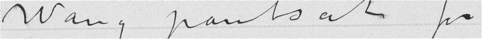Wang pantsat for
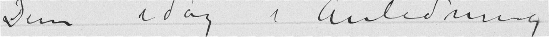Dem idag i Anledning
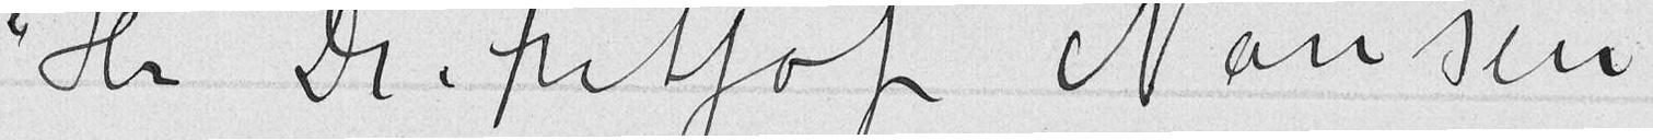Hr Dr. Fritjof Nansen
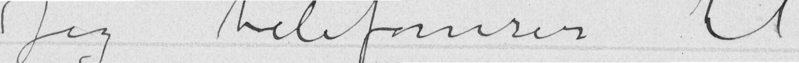Jeg telefonerer til
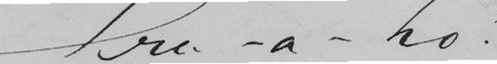Tra-a-ho!
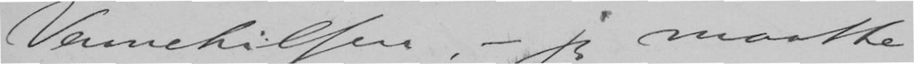Vennehilsen, - jeg maatte
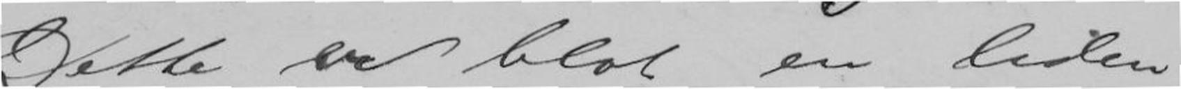Dette er blot en liden
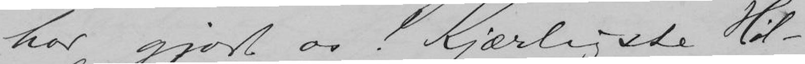har gjort os! Kjærligste Hil-
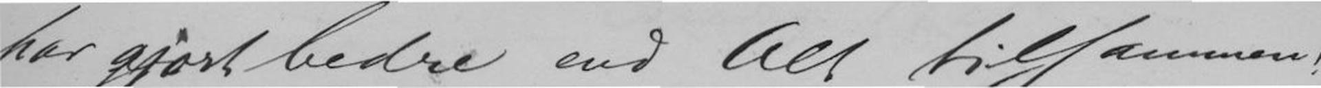har gjort bedre end Alt tilsammen!
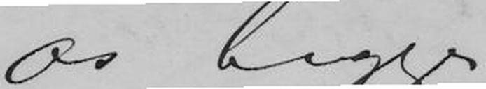os begge.
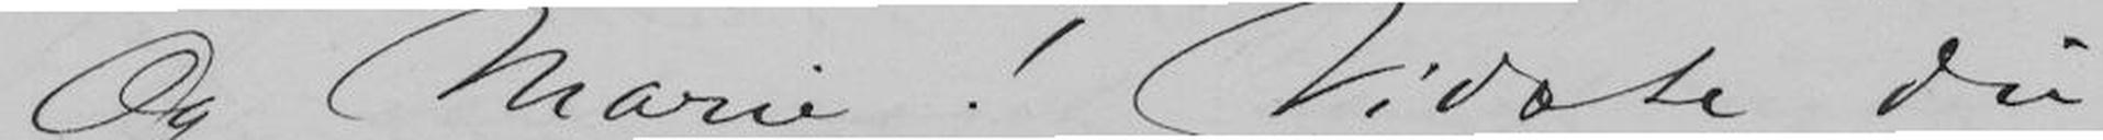Og Marie! Vidste Du
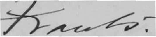Frants.
